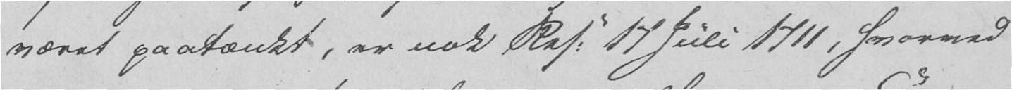været paatænkt, er nok Res. 17 Juli 1711, hvorved
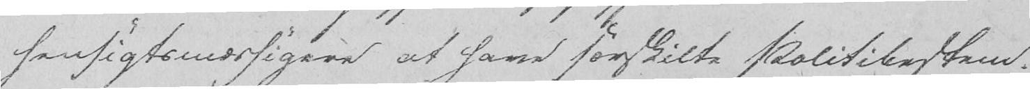hensigtsmæssigere at have særskilte Politibestem-
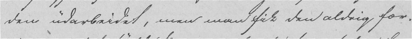den udarbeidet, men man fik den aldrig fær-
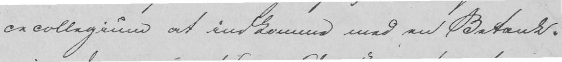cecollegium at indkomme med en Betænk-
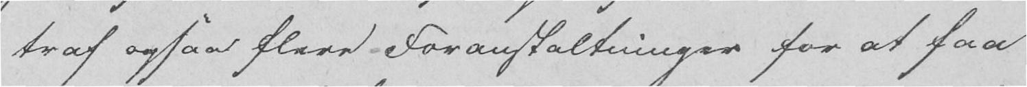traf ogsaa flere Foranstaltninger for at faa
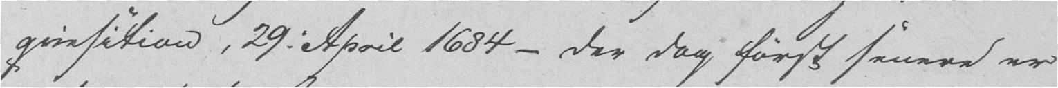qvisition, 29. April 1684 - der dog først senere er
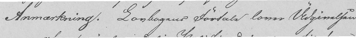Anmærkning. Lovbogens Fortale lover Udgivelsen
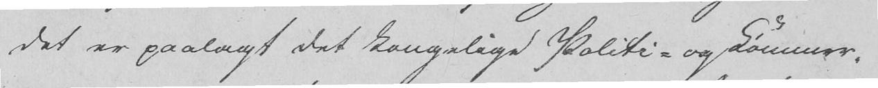det er paalagt det kongelige Politi- og Commer-
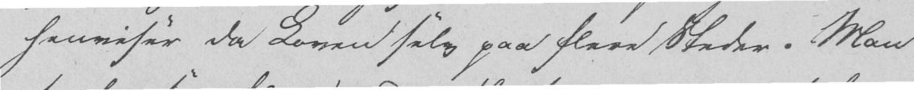henviser da Loven selv paa flere Steder. Man
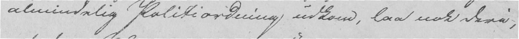almindelig Politiordning udkom, laa nok deri,
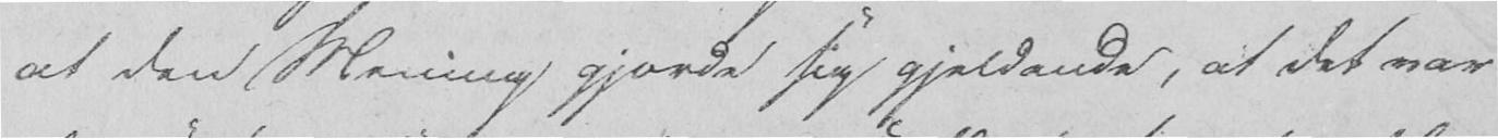at den Mening gjorde sig gjeldende, at det var
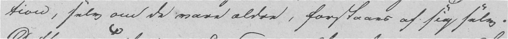tion, selv om de vare ældre, forstaaes af sig selv.
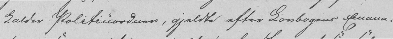kalder Politiuordner, gjeldte efter Lovbogens Emana-
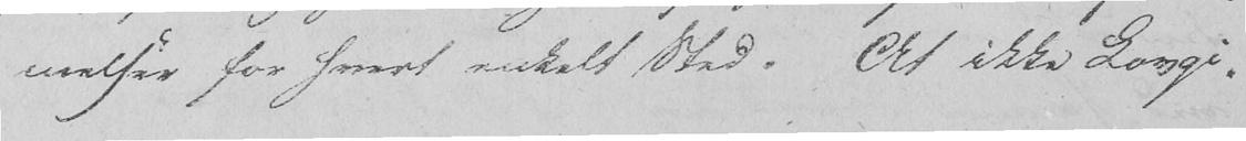melser for hvert enkelt Sted. At ikke Lovgi-
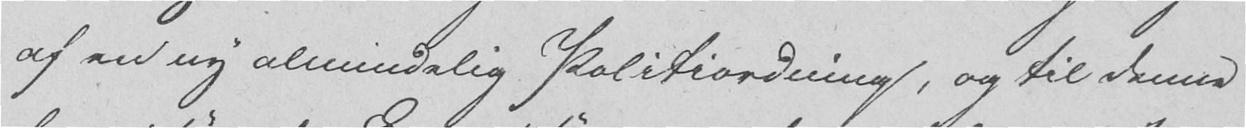af en ny almindelig Politiordning, og til denne
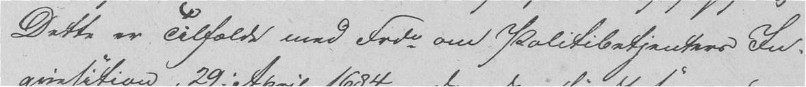Dette er Tilfælde med Frdn om Politibetjenters In-
